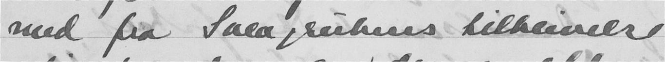med fra Sveagrubens tilblivelse
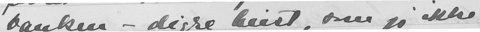banken - digre beist som jeg ikke
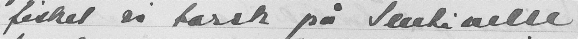fisket vi torsk på Sentinelle
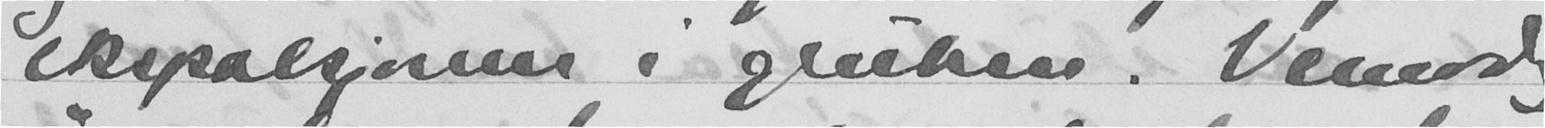eksplosjonen i gruben. Vemodig
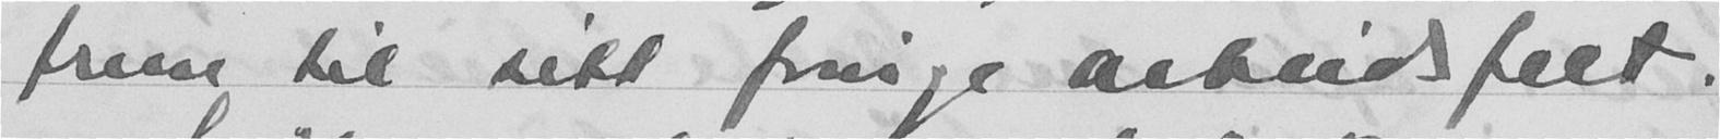frem til sitt forrige arbeidsfelt.
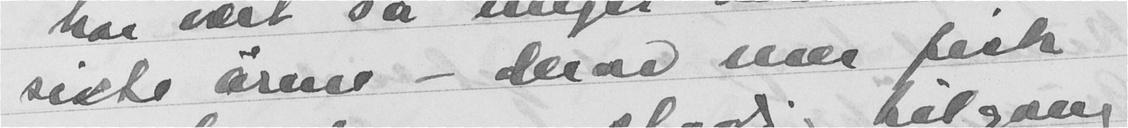siste årene - derav mer fisk
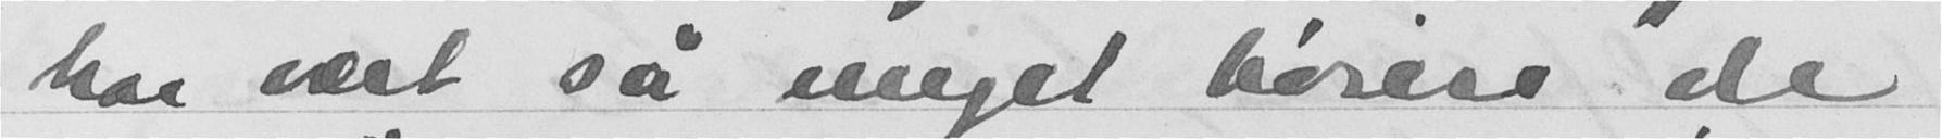har vært sa meget høiere de
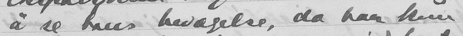å se hans bevægelse, da han kom
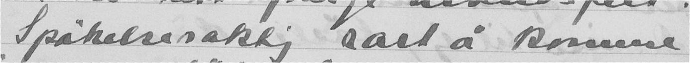Spøkelsesaktig rart å komme
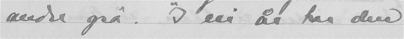andre også. I ni år har den
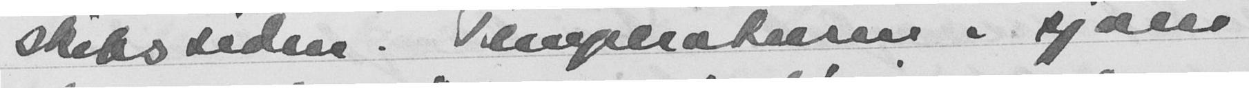skibssiden. Temperaturen i sjøen
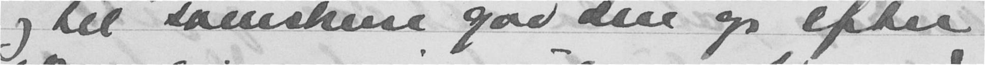og til svenskense gav den op efter
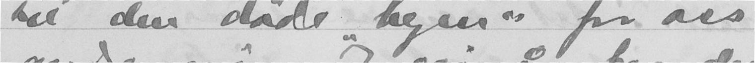til den døde "byen" for oss
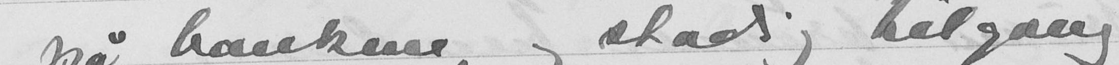på bankene og stadig tilgang
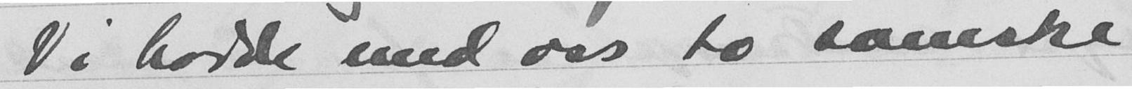Vi hadde med oss to svenske
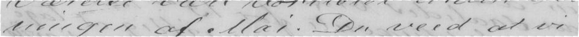ningen af Mai. Du veed at vi -
